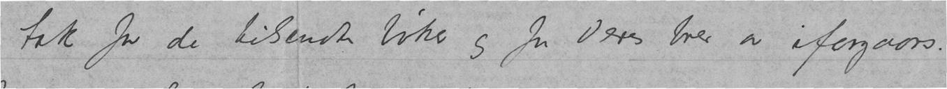tak for de tilsendte bøker og for Deres brev av iforgaars.
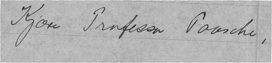Kjære Professor Paasche,
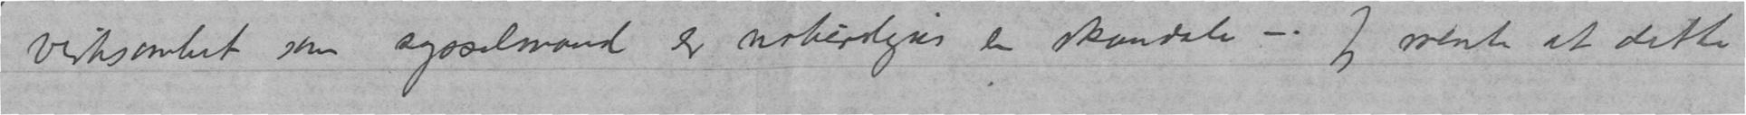virksomhet som sysselmand er naturligvis en skandale –. Jeg mente at dette
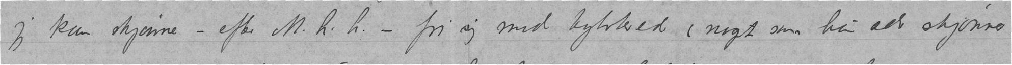jeg kan skjønne – efter M.L.L. – fri sig med byttered (noget som hun selv skjønner
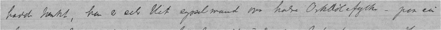hadde tænkt, han er selv blit sysselmand over halve Orkdølefylke – paa sin
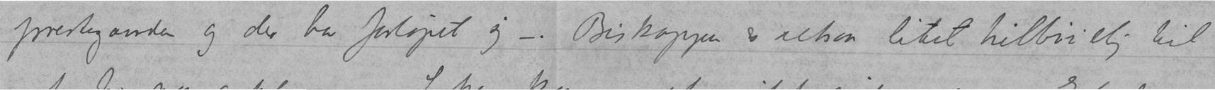prestegaarden og der har forløpet sig –. Biskoppen er altsaa litet tilbøielig til
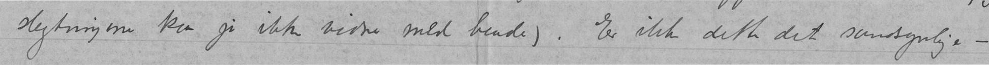slegtningerne kan jo ikke vidne med hende). Er ikke dette det sandsynlige –
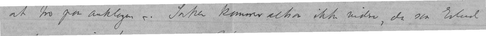at tro paa anklagen –. Saken kommer altsaa ikke videre, da saa Erlend
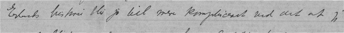Erlends historie blir jo litt mere kompliceret ved det at jeg
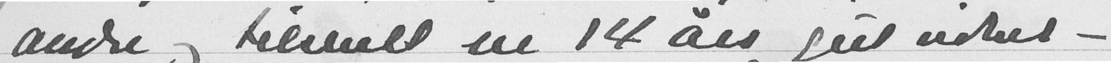andre og tilslutt en 14 års gut vidnet -
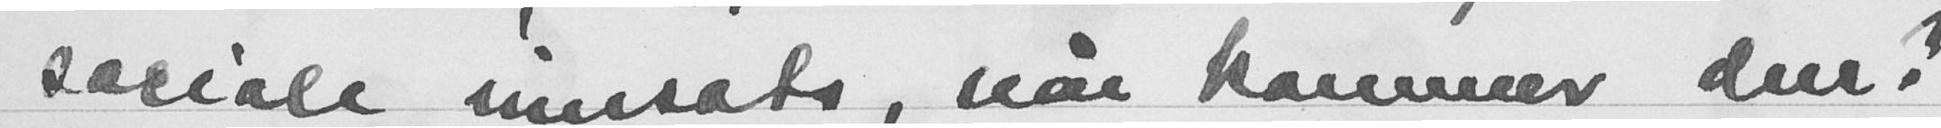sosiale innsats, når kommer den?
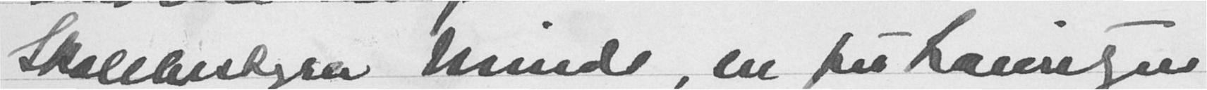Skolebestyrer Minde, en fru Haresoingen
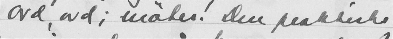Ord ord; møter. Den praktisk-
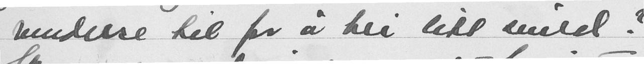vendelse til for å bli litt snild?
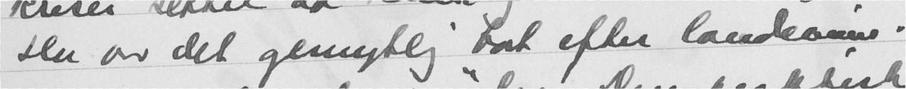Her var det geringtlig bot efter landeveie.
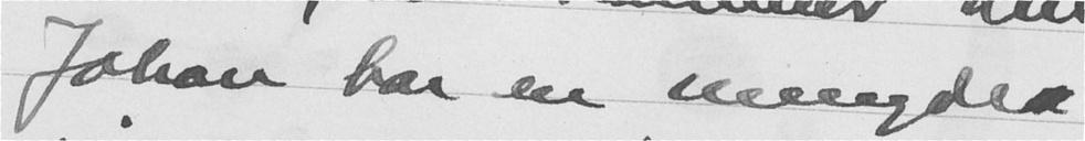Johan har en mengde
